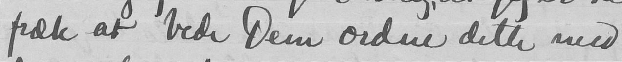fræk at bede Dem ordne dette med
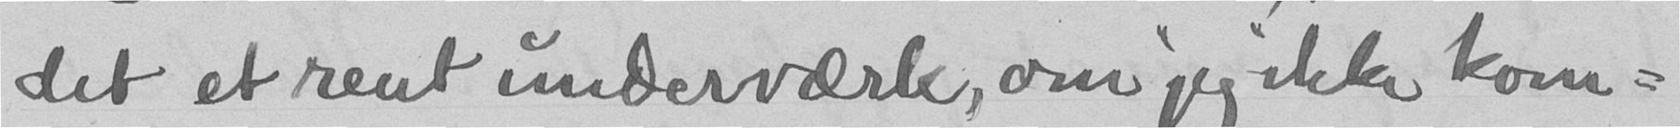det et rent underværk, om jeg ikke kom-
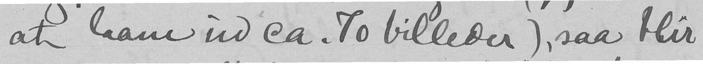at laane ud ca. to billeder), saa blir
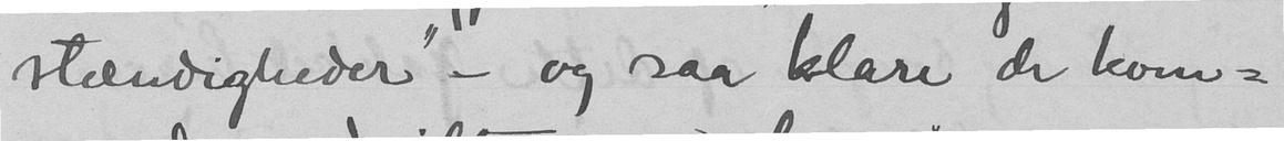stændigheder" - og saa klare de kom-
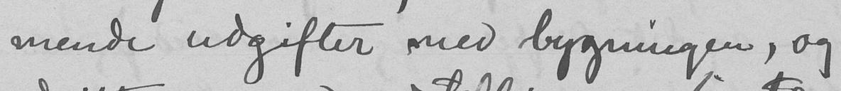mende udgifter med bygningen, og
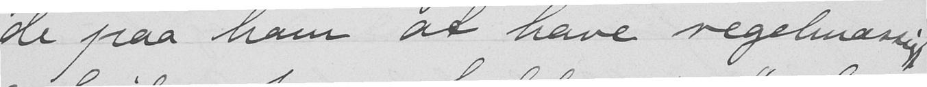de paa ham at have regelmæssig
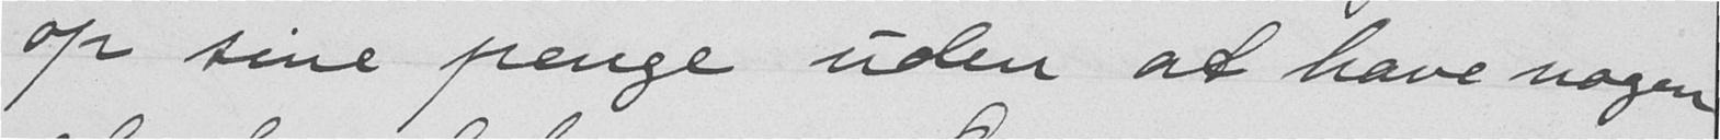op sine penge uden at have nogen
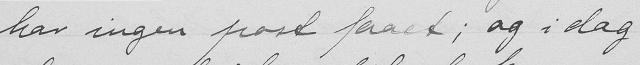har ingen post faaet; og i dag
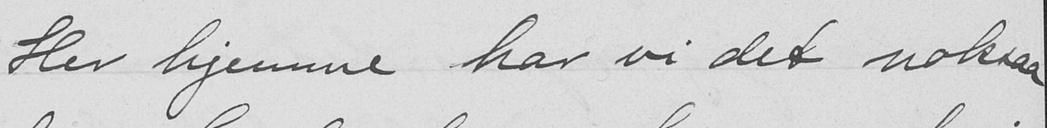Her hjemme har vi det noksaa
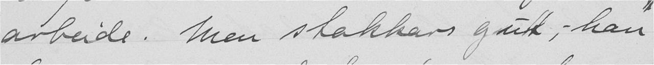arbeide. Men stakkars gut, - han
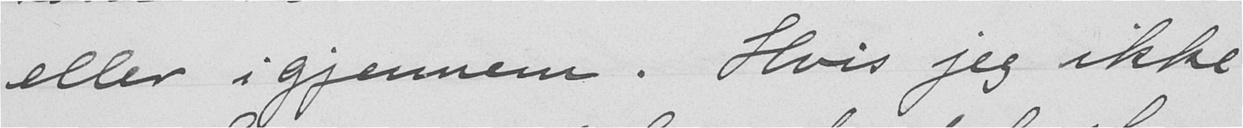eller igjennem. Hvis jeg ikke
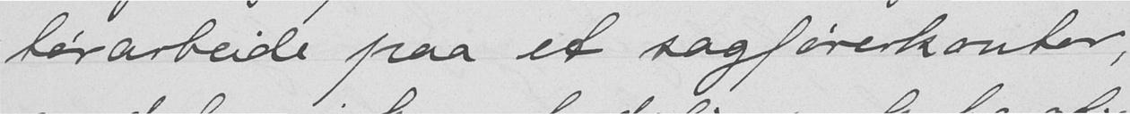tørarbeide paa et sagførerkontor,
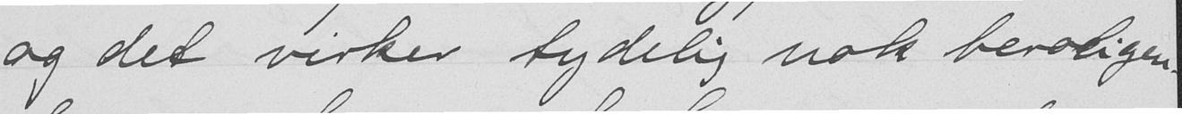og det virker tydelig nok beroligen-
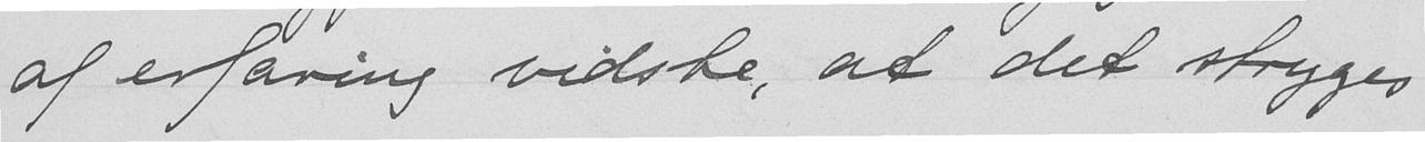af erfaring vidste, at det stryges
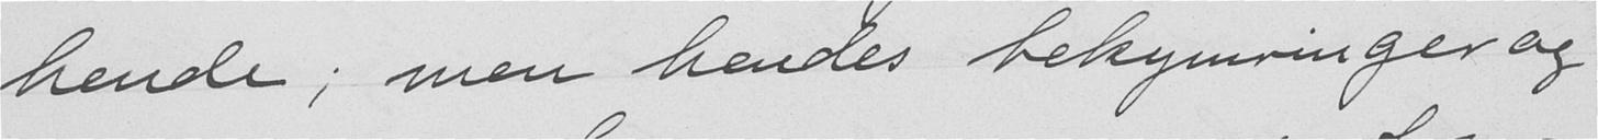hende; men hendes bekymringer og
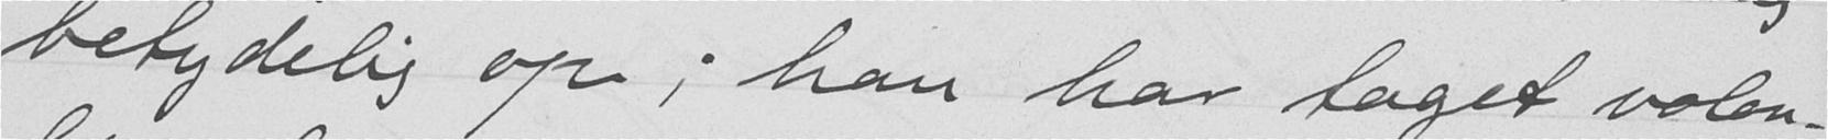betydelig op; han har taget volon-
